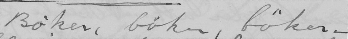Böker, böker, böker –
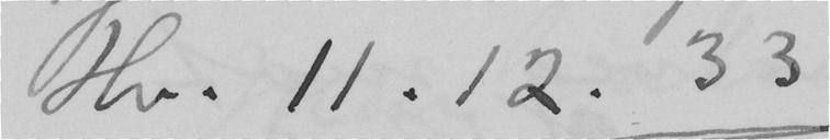Hv. 11.12.33
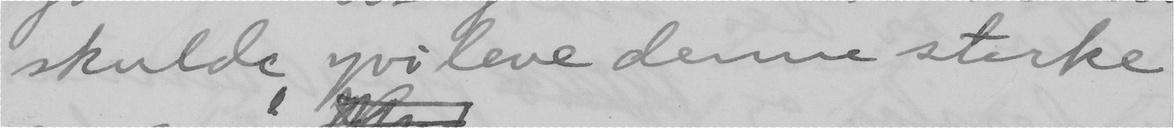skulde yvileve denne sterke
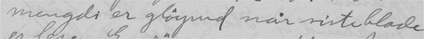mengdi er glöymd når siste blade
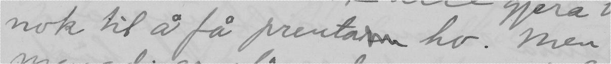nok til å få prenta ho. Men
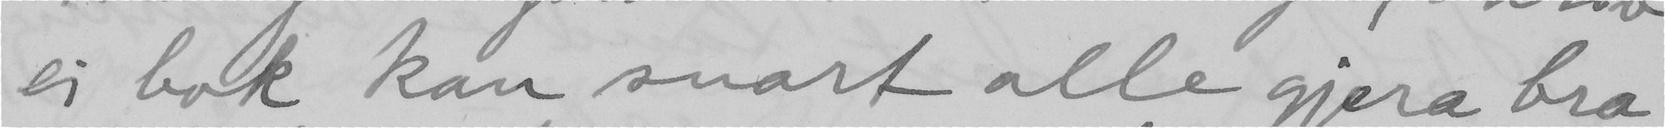ei bok kan snart alle gjera bra
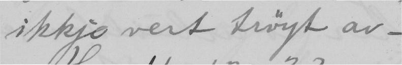ikkje vert tröyt av.
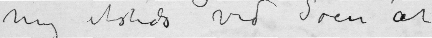mig etsteds ved Søen at
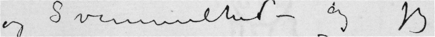og Svimmelhed – og jeg
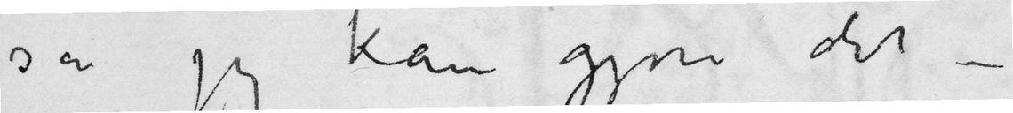så jeg kan gjøre det –
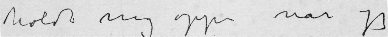holdt mig oppe når jeg
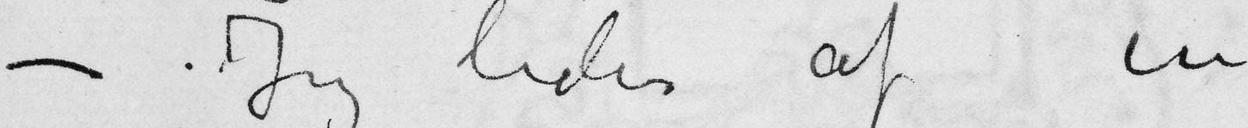– Jeg lider af en
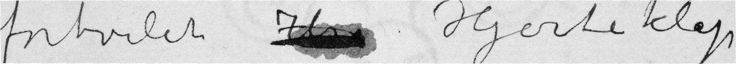fortvilet Hjerteklap
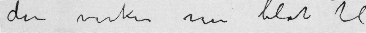den virker nu blot til
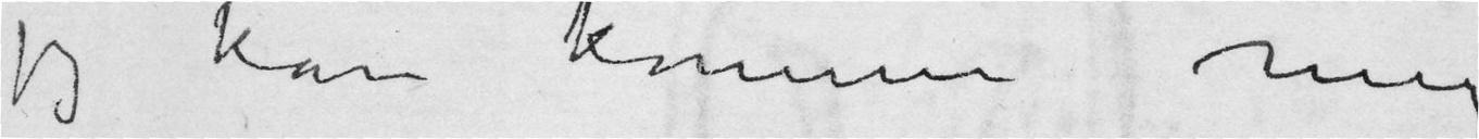jeg kan komme mig
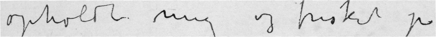opholdt mig og frisket på
